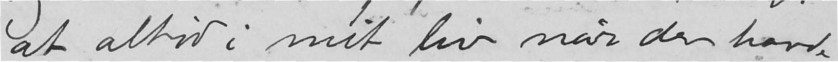at altid i mit liv når der havde
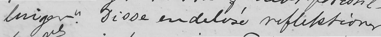linger". Disse endeløse reflektioner
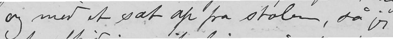og med et sat op fra stolen, så jeg
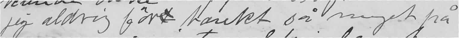jeg aldrig ført tænkt så meget på
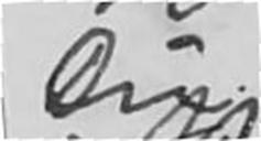Du
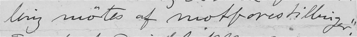ling møtes af motforestillinger."
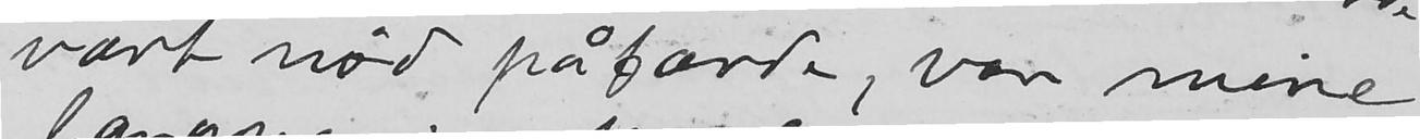vært nød påfærde, var mine
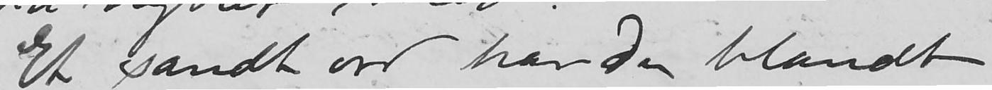Et sandt ord hav Du blandt
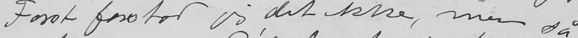Først forstod jeg det ikke, men så
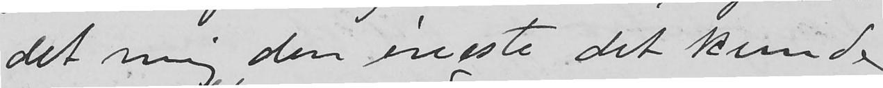det mig den eneste det kunde
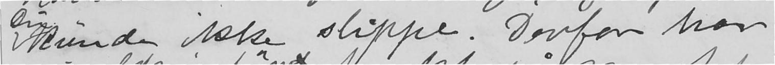kunde ikke slippe. Derfor har
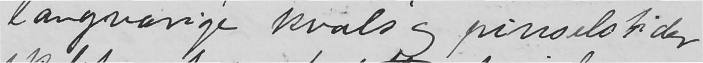langvarige kvals og pinselstider
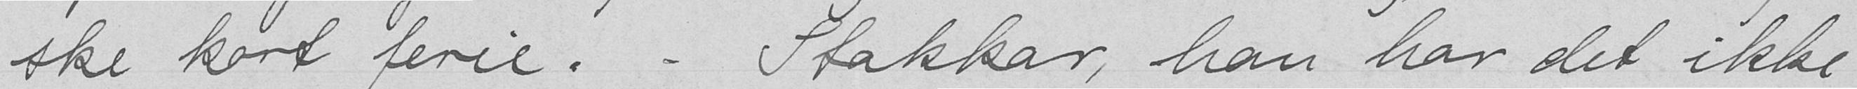ske kort ferie. - Stakkar, han har det ikke
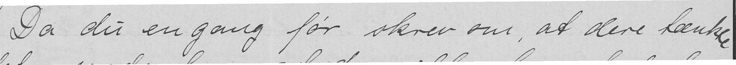Da du en gang før skrev om, at dere tænkte
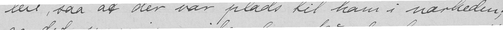leie, saa at der var plads til ham i nærheden,
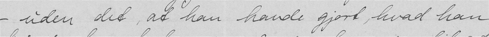- uden det, at han havde gjort, hvad han
